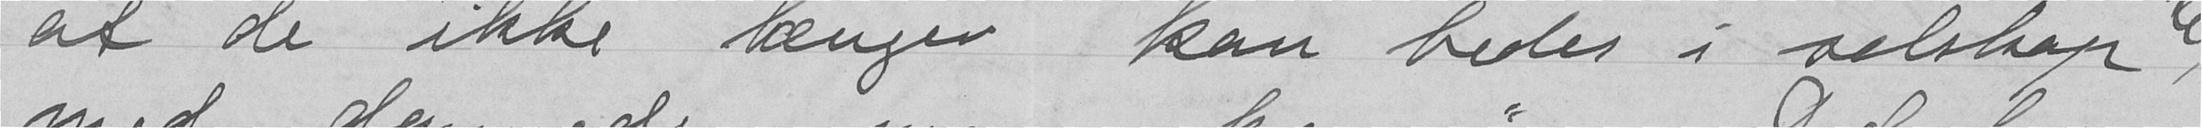at de ikke længer kan bedes i selskap
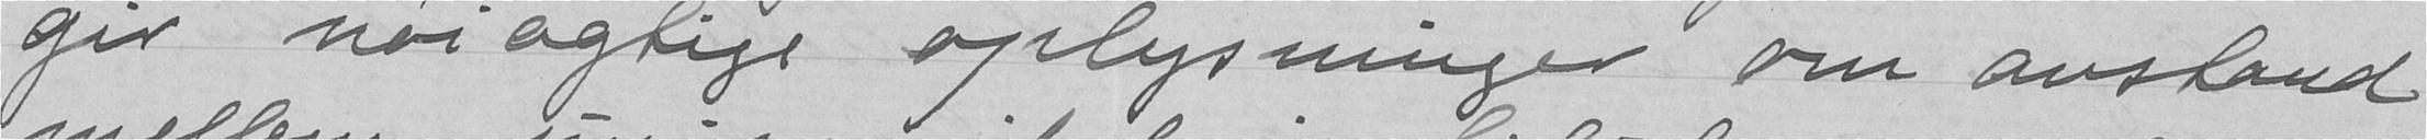gir nøiagtige oplysninger om avstand
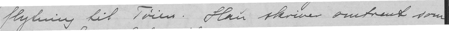flytning til Tøien. Han skriver omtrent som
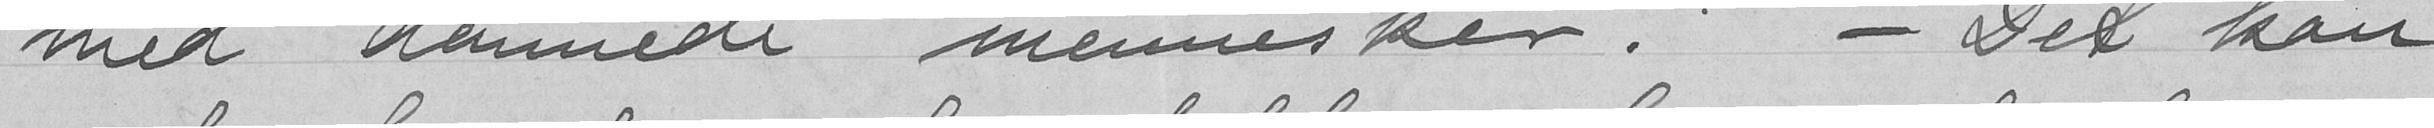med dannede mennsker. - Det kan
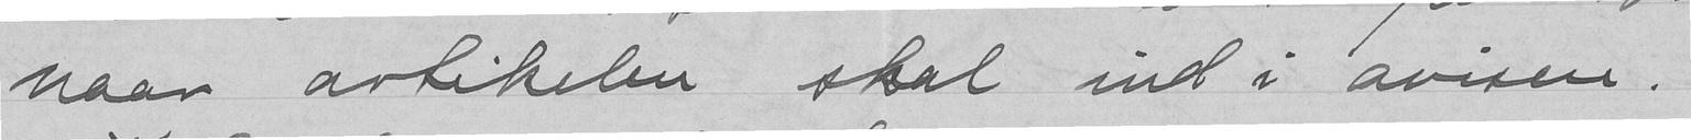naar artikelen skal ind i avisen.
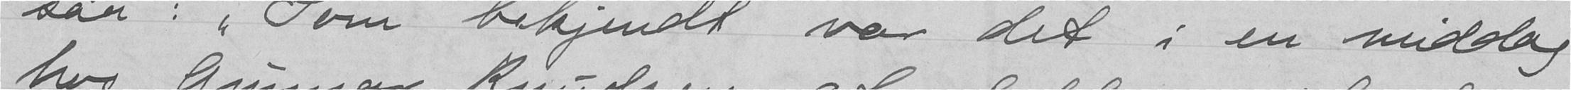saa: Som bekjendt var det i en middag
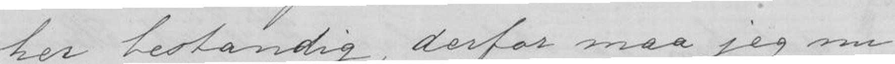her bestandig, derfor maa jeg nu
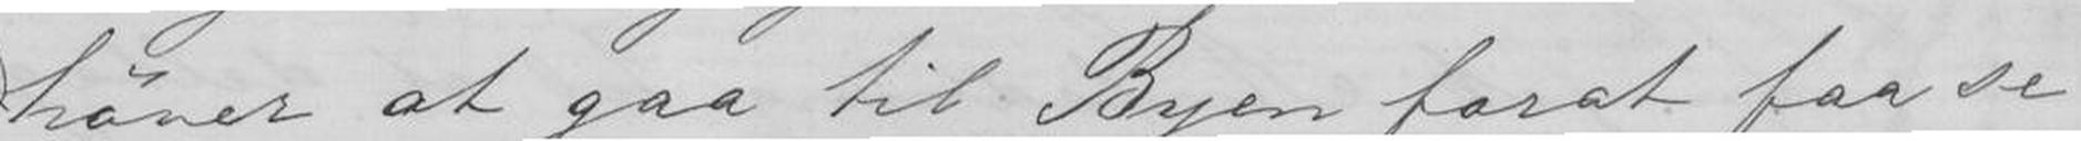høver at gaa til Byen forat faa se
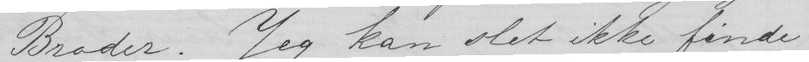Broder. Jeg kan slet ikke finde
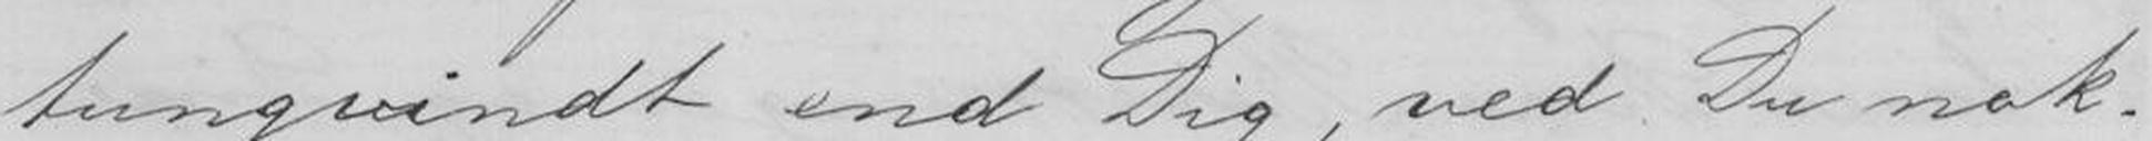tungvindt end Dig, ved Du nok.
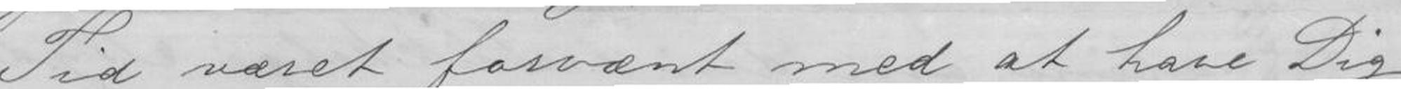Tid været forvænt med at have Dig
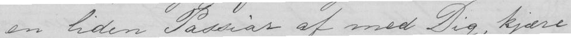en liden Passiar af med Dig, kjære
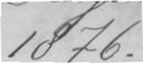1876.
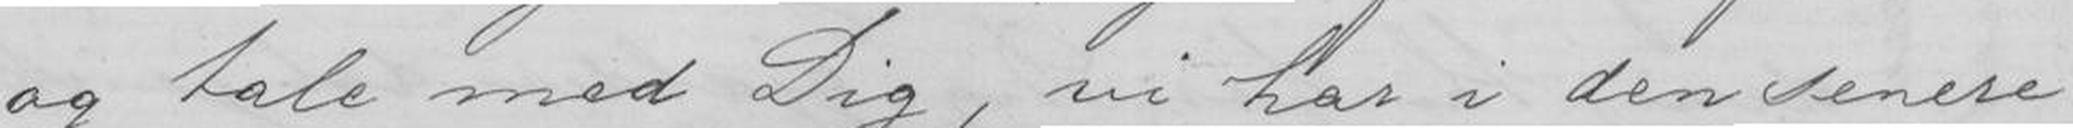og tale med Dig, vi har i den senere
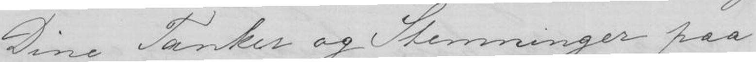Dine Tanker og Stemninger paa
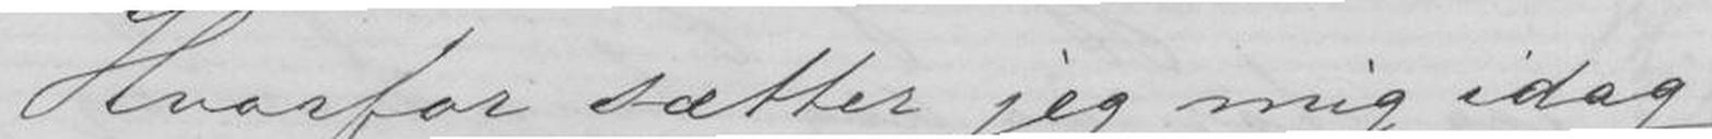Hvorfor sætter jeg mig idag
